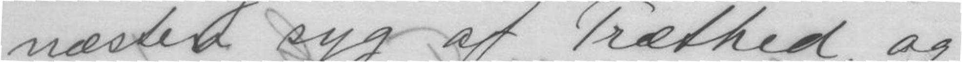næsten syg af Træthed, og
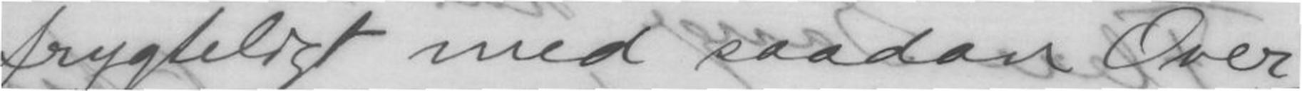frygteligt med saadan Over-
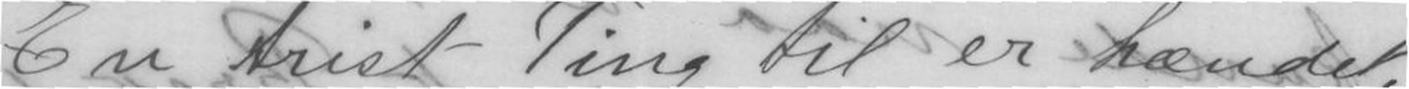En trist Ting til er hændet
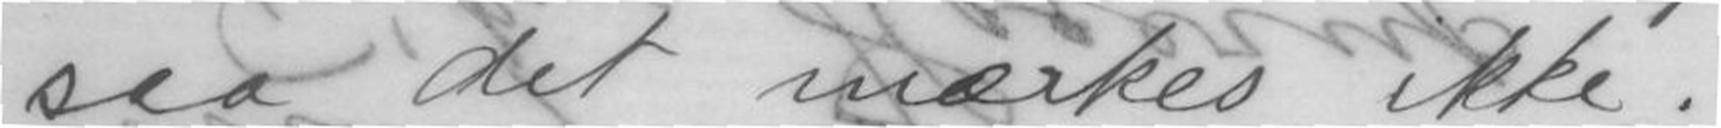saa det mærkes ikke.
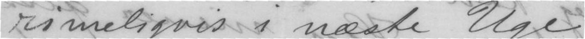rimeligvis i næste Uge
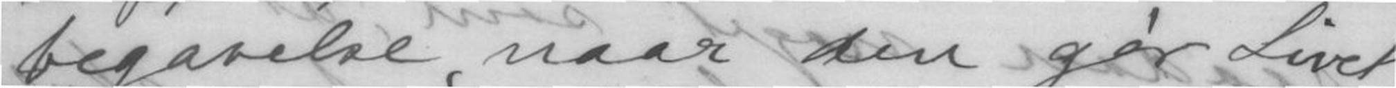begavelse, naar den gjør Livet
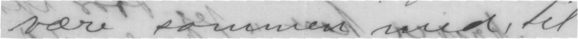være sammen med, til
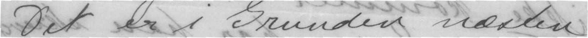Det er i Grunden næsten
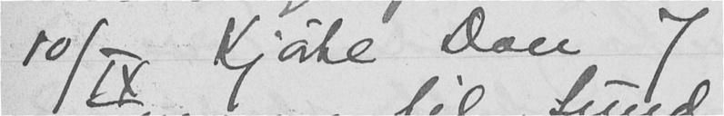10/IX Kjørte Don 7
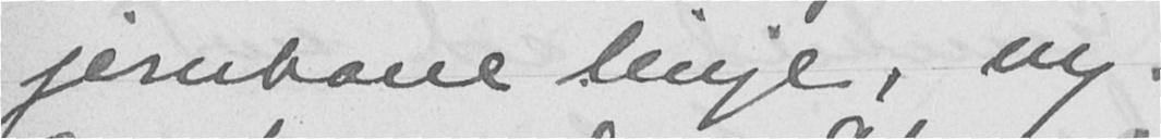jernbanelinje, ny.
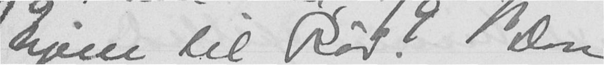hjem til Rød. Don
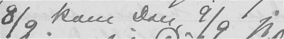8/9 kom Don, 9/9 jeg
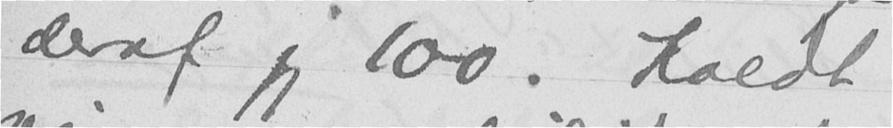deraf jeg 100. Koldt
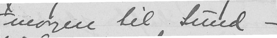morgen til Sund -
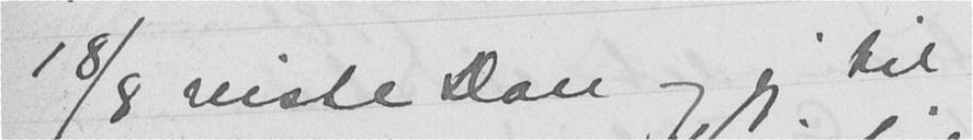18/8 reiste Don og jeg til
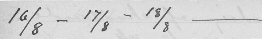16/8 - 17/8 - 18/8 -
